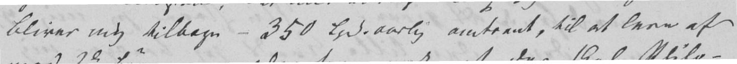Bliver mig tilbage – 350 Spd. aarlig omtrent, til at leve af
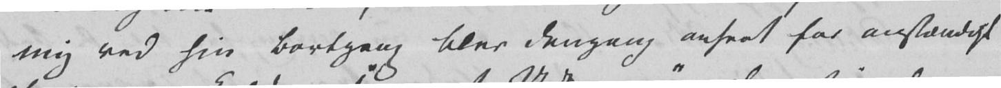mig ved sin Bortgang blev dengang anseet for anstændigt
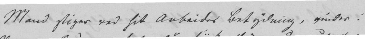Mand stiger ved sit Arbeides Betydning, vinder i
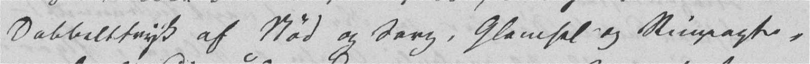Dobbelttryk af Nød og Sorg, Glemsel og Ringeagt,
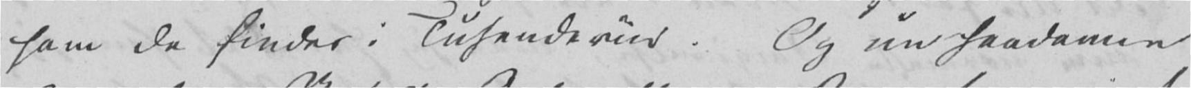som de findes i Tusendeviis. Og nu saadanne
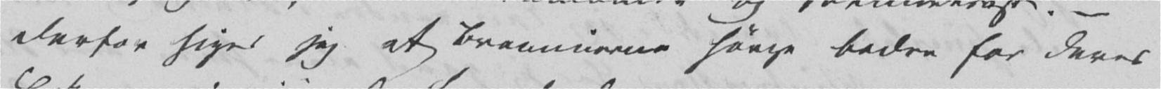Derfor siger jeg at Braminerne sørge bedre for deres
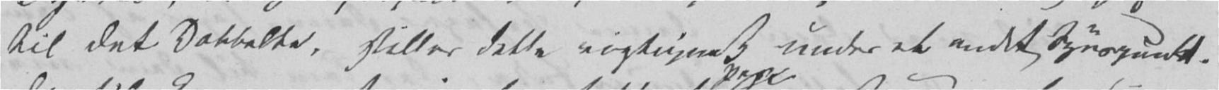til det Dobbelte, stiller dette rigtignok under et andet Synspunkt.
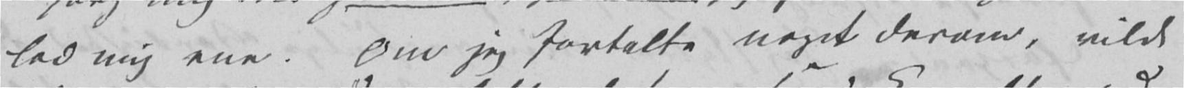lod mig ene. Om jeg fortalte noget derom, vilde
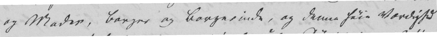og Moder, Borger og Borgerinde, og denne høie Værdighed
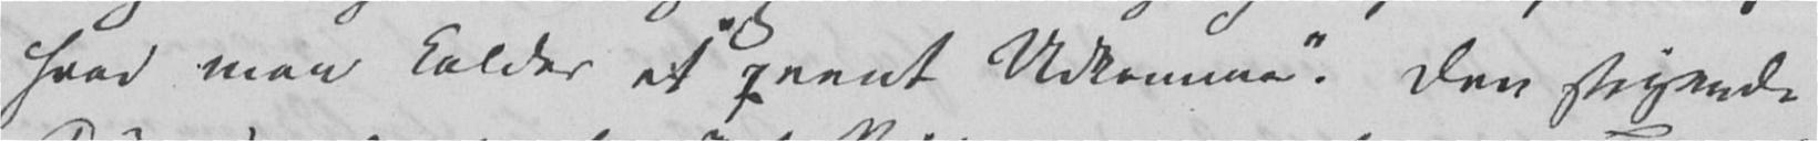hvad man kalder et «peent Udkomme». Den stigende
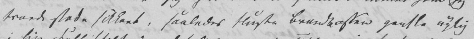troede stode sikkert, saaledes slugte Brandkassen ganske nylig
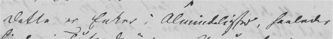Dette er Enker i Almindelighed, saaledes
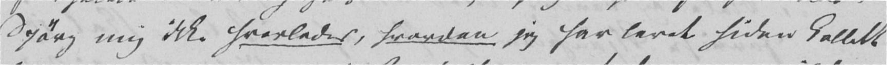Spørg mig ikke hvorledes, hvordan jeg har levet siden Collett
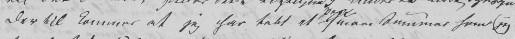Der til kommer at jeg har tabt et Par smaae Summer som jeg
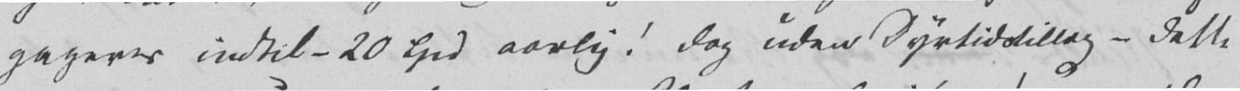gageres indtil – 20 Spd aarlig! dog uden Dyrtidstillæg – dette
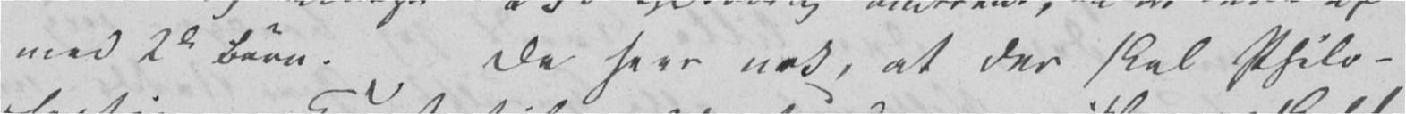med 2de Børn. De seer nok, at der skal Philo-
